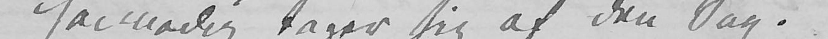høimodig tager sig af den Sag.
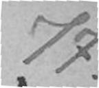77.
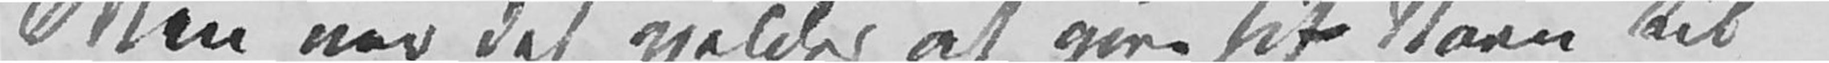Men når det gjelder at give sit Navn til
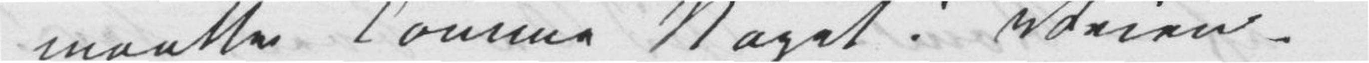maatte komme Noget i Veien.
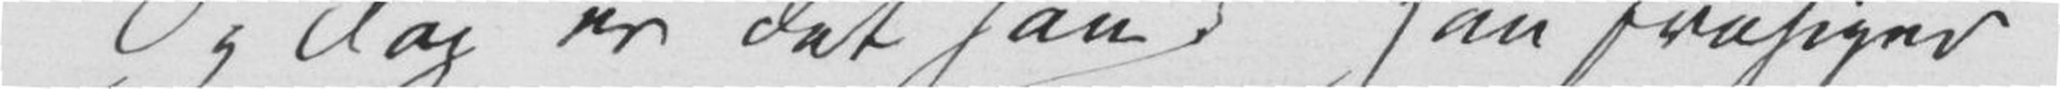Og dog er det han. Han frasiger
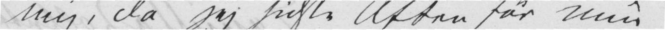mig, da jeg sidste Aften før min
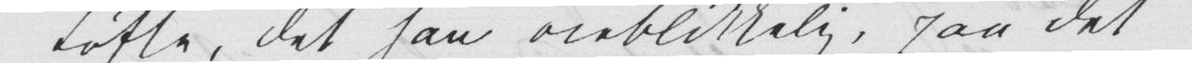Løfte, det han øieblikkelig, paa det
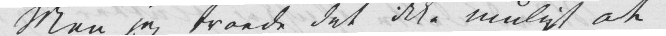Men jeg troede det ikke muligt at
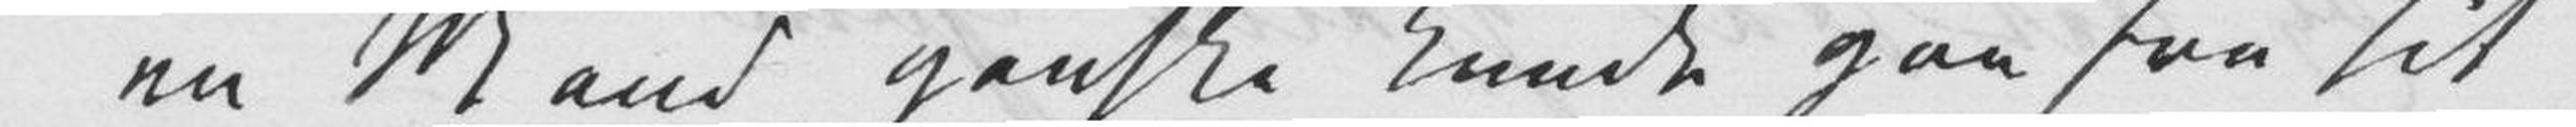en Mand ganske kunde gaa fra sit
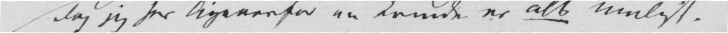Dog jeg ser ligeoverfor en Kvinde er alt muligt.
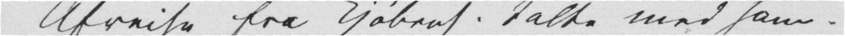Afreise fra Kjøbenh. talte med ham.
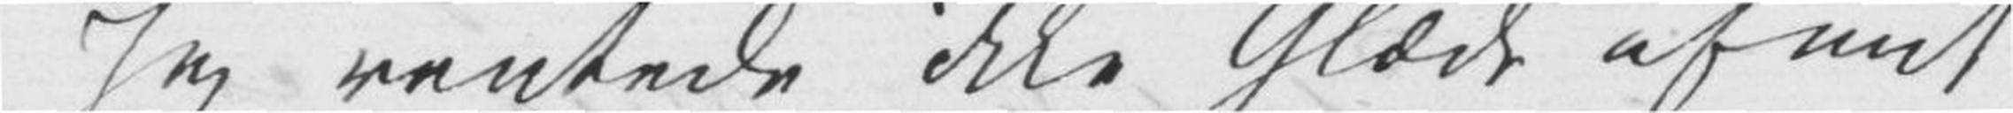Jeg ventede ikke Glæde af mit
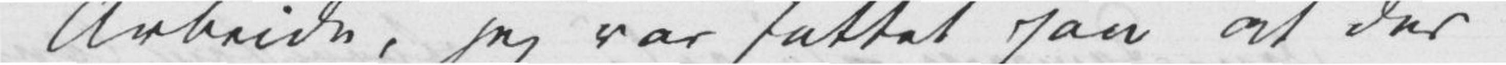Arbeide, jeg var fattet paa at der
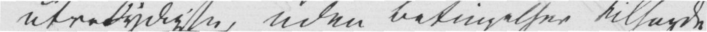utvetydigste, uden Betingelser tilsagde
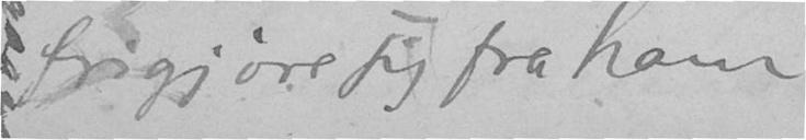frigjøre jeg fra ham
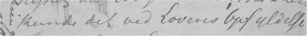kunde det ved Lovens Opfyldelse
